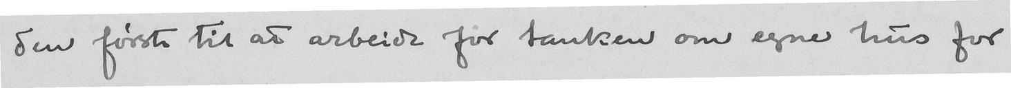den første til at arbeide for tanken om egne hus for
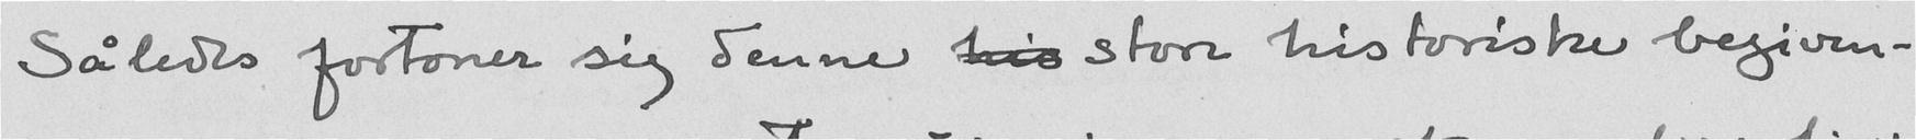Således fortoner sig denne his store historiske begiven-
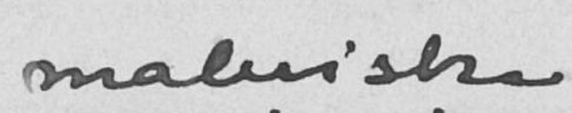maleriske
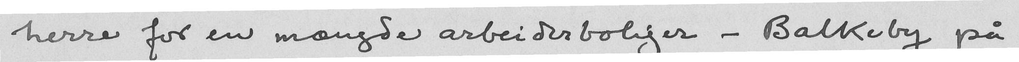herre for en mængde arbeiderboliger - Balkeby på
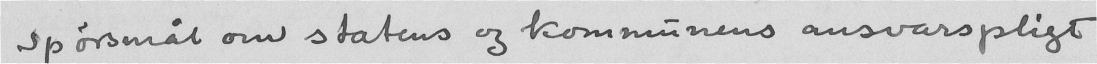spørsmål om statens og kommunens ansvarspligt
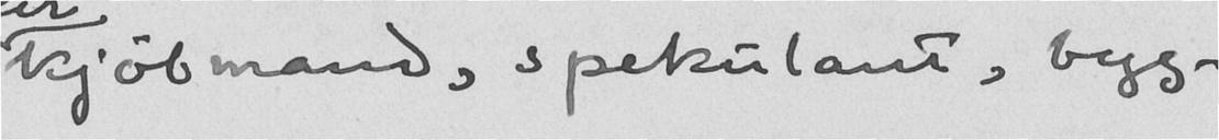kjøbmand, spekulant, byg-
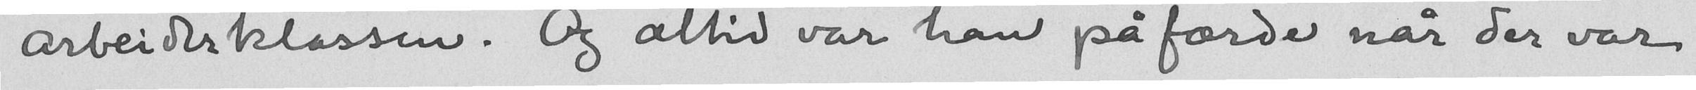arbeiderklassen. Og altid var han på færde når der var
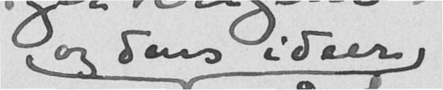og dens ideer
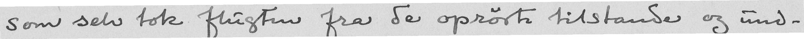som selv tok flugten fra de oprørte tilstande og und-
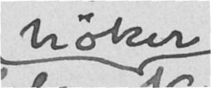høker
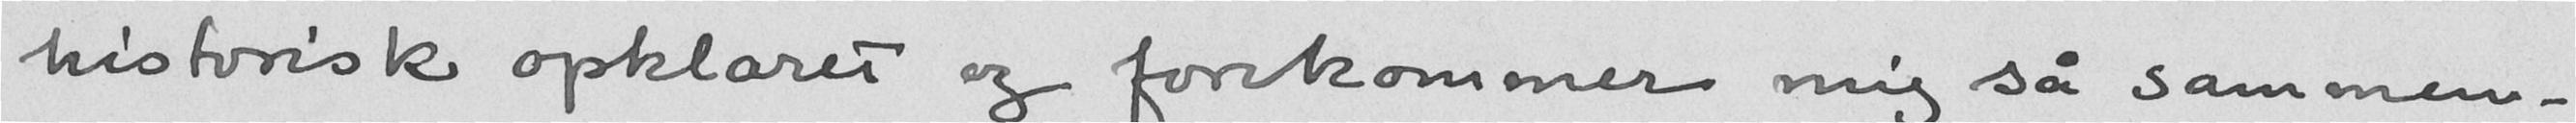historisk opklaret og forekommer mig så sammen-
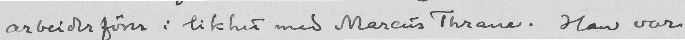arbeiderfører i likket med Mareus Thrane. Han var
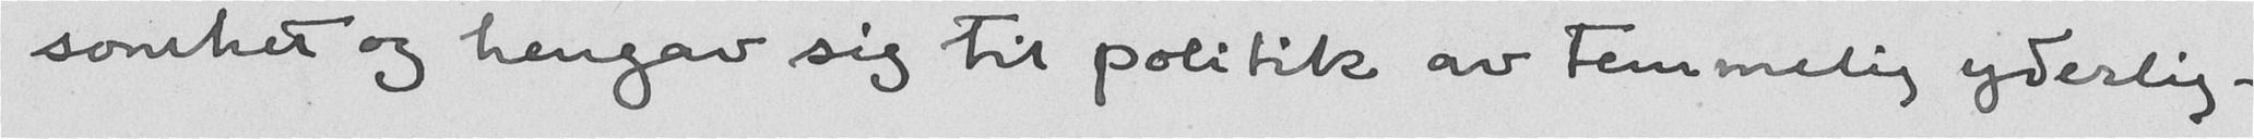somhet og hengav sig til politik av Temmelig yderlig-
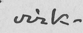virk-
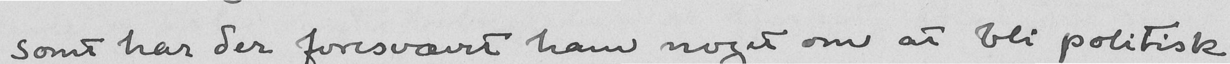somt har der foresvævet ham noget om at bli politisk
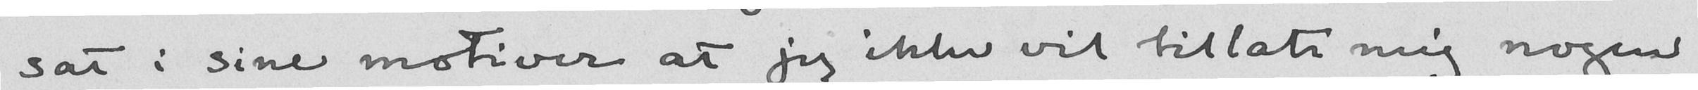sat i sine motiver at jeg ikke vil tillate mig nogen
